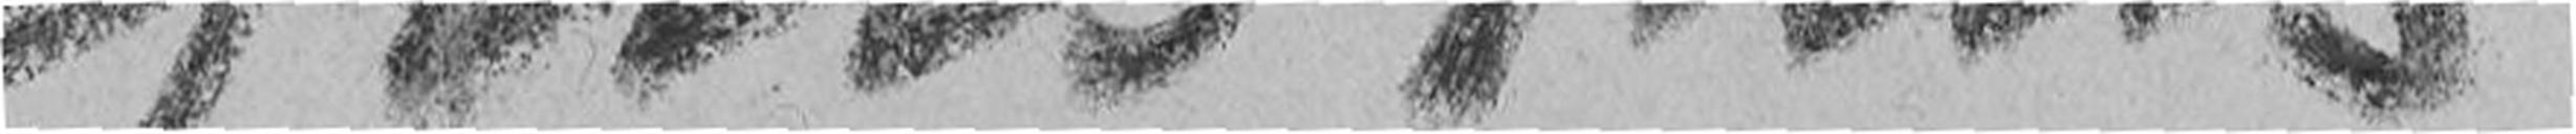Jens Thiis
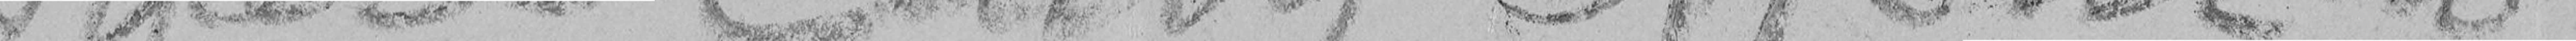Kjære Cally Monrad
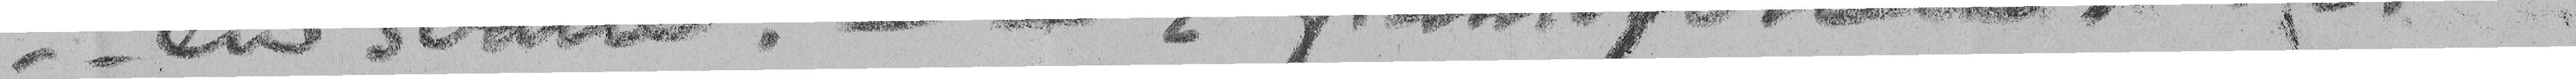«–en svane» – – i gramofonen! Vor
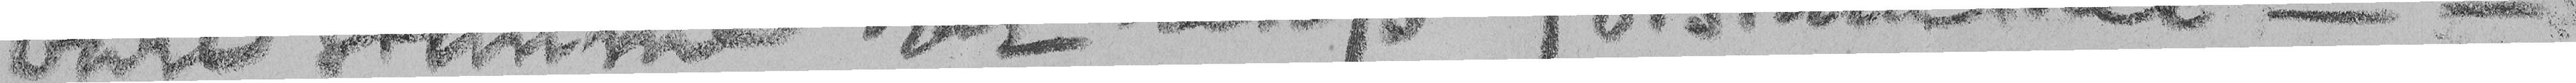bare stemme har netop forstummet – –
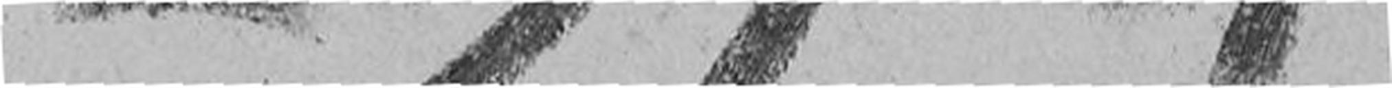21/1 11
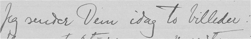Jeg sender Dem idag to billeder:
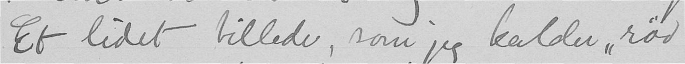Et lidet billede, som jeg kalder "rød
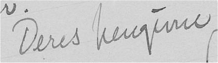Deres hengivne
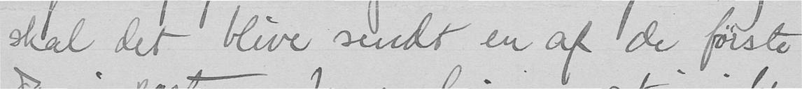skal det blive sendt en af de første
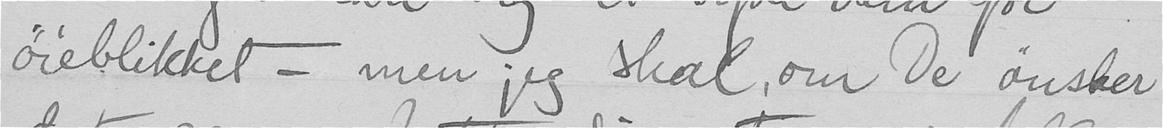øieblikket - men jeg skal, om De ønsker
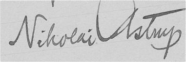Nikolai Astrup
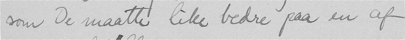som De maate like bedre paa en af
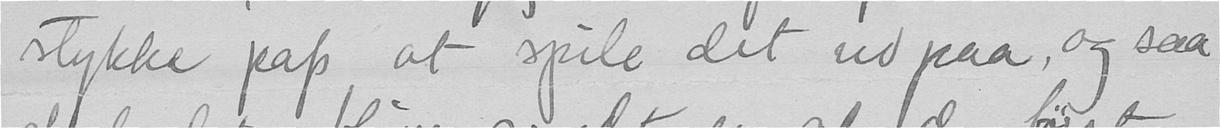stykke pap at spile det ud paa, og saa
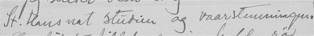St.Hans nat studien og vaarstemningen.
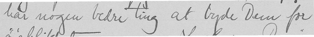har nogen bedre ting at byde Dem for
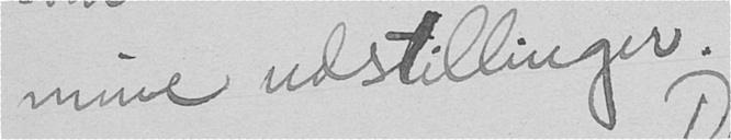mine udstillinger.
